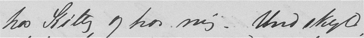hos Kitty og hos mig. Undskyld
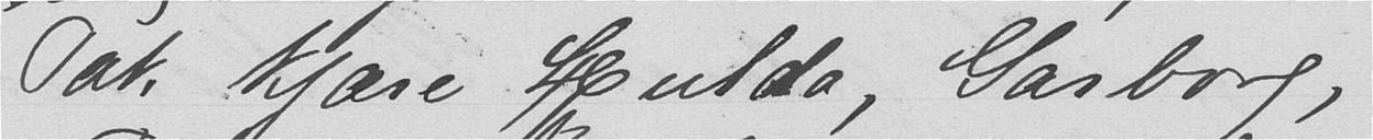Tak Kjære Hulda, Garborg,
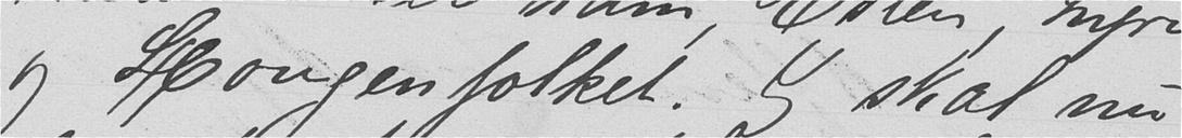og Hougenfolket. Jeg skal nu
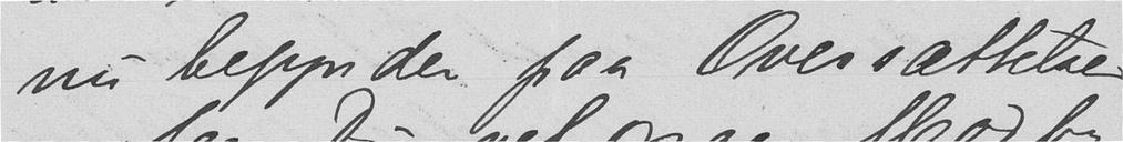nu begynder paa Oversættelse
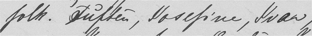folk. Tuften, Josefine, Ivar
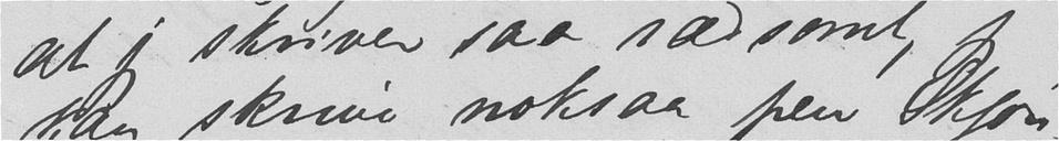kan skrive noksaa hen Skjøn-
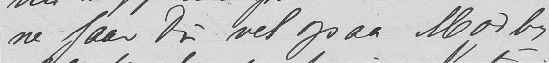ne faar Du vel ogsaa Modby-
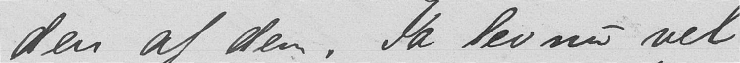den af dem. Ja lev nu vel
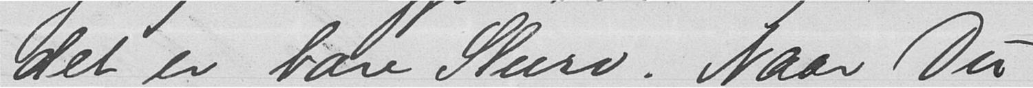det er bare Slurv. Naar Du
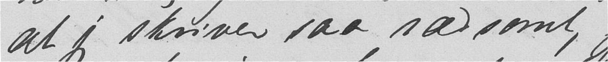at jeg skriver saa rædsomt,
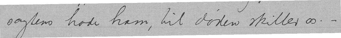sagtens hade ham, til døden skiller os. -
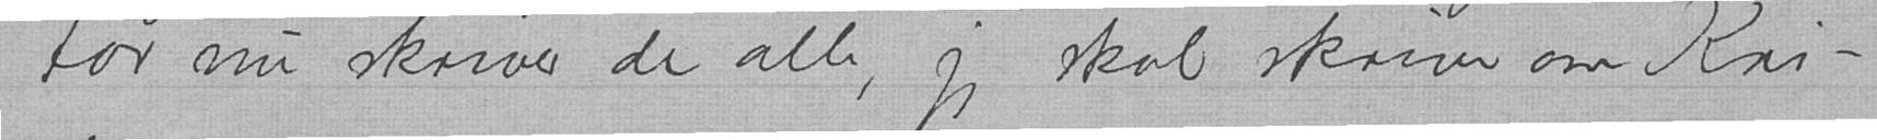For nu skriver de alle, jeg skal skrive om Kri-
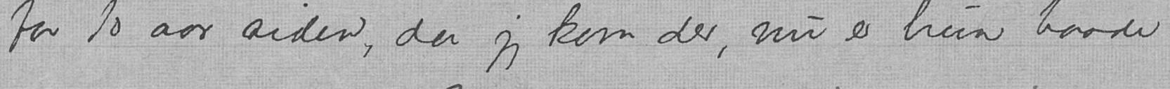for 10 aar siden, da jeg kom der, nu er hun baade
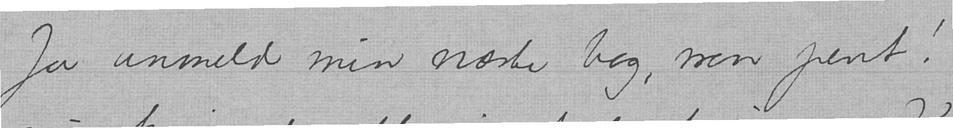Ja anmeld min næste bog, men pent!
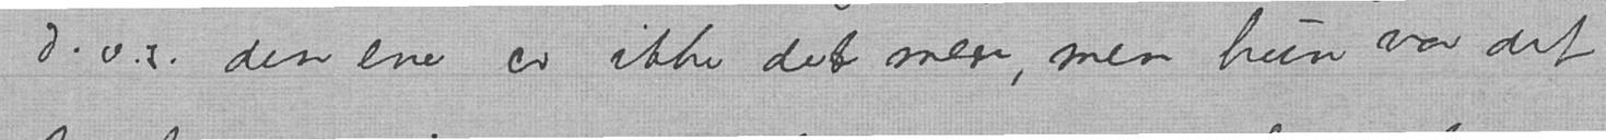d.v.s. den ene er ikke det mere, men hun var det
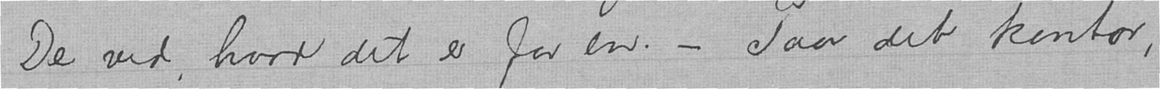De ved, hvad det er for en. - Paa det kontor,
